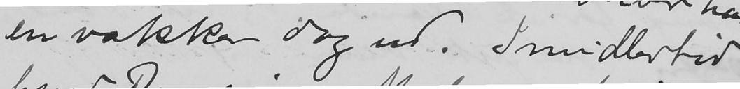en vakker dag ud. Imidlertid
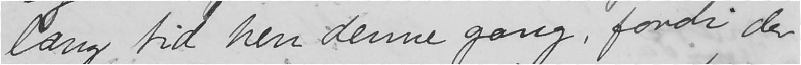lang tid hen denne gang, fordi der
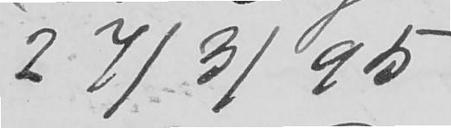27/3/95
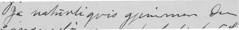Ja naturligvis gjemmer Du
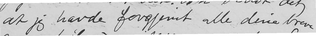at jeg havde forgjemt alle dine breve
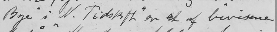Bye" i N. Tidskrift "er et af bevisene
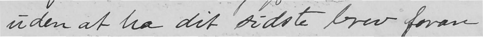uden at ha dit sidste brev foran
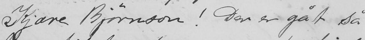Kjære Bjørnson! Den er gåt så
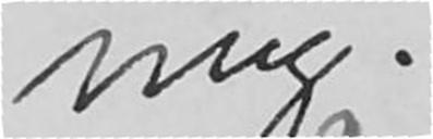mig.
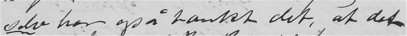selv har også tænkt det, at det
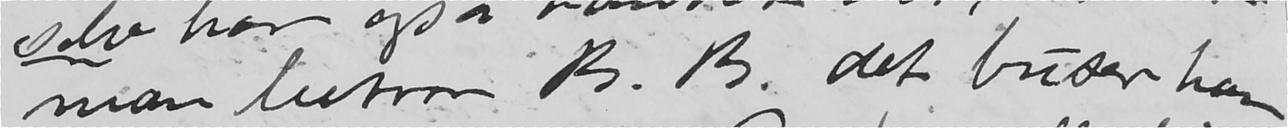man betror B.B. det buser han
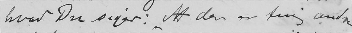hvad Du siger: "At der er ting andre
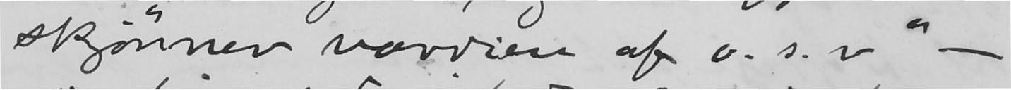skjønnev værdien av o.s.v" -
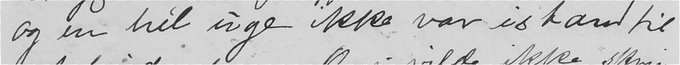og en hél uge ikke var istand til
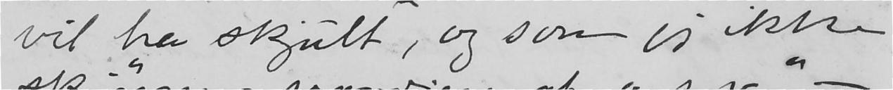vil ha skjult, og som jeg ikke
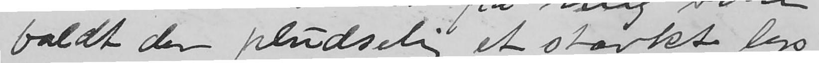faldt der pludselig et stærkt lys
